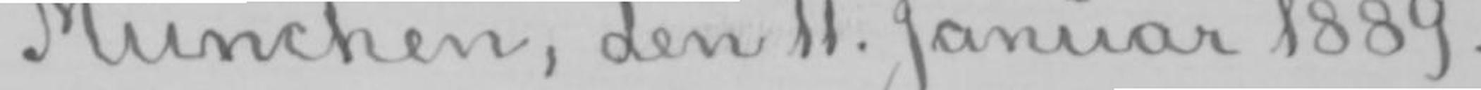München, den 11. Januar 1889.
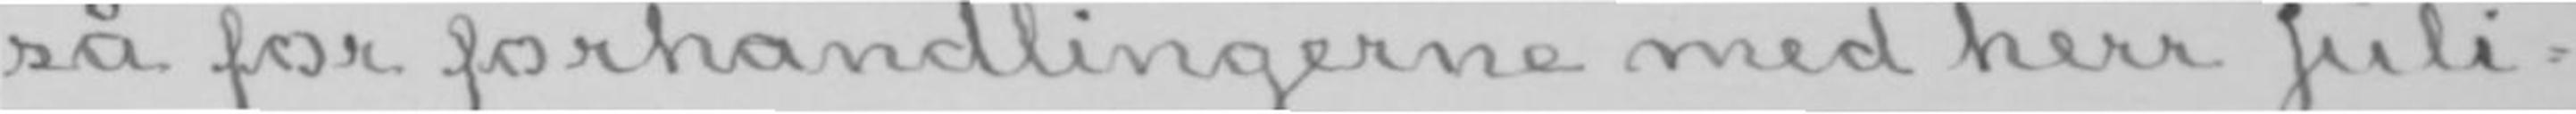så for forhandlingerne med herr Juli-
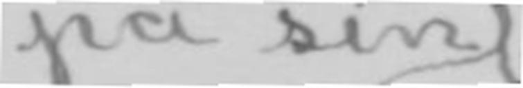på sin
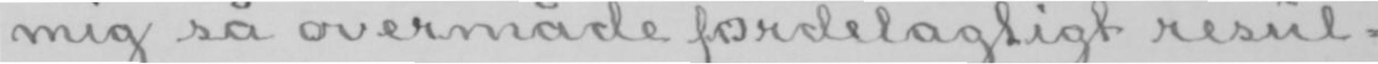mig så overmåde fordelagtigt resul-
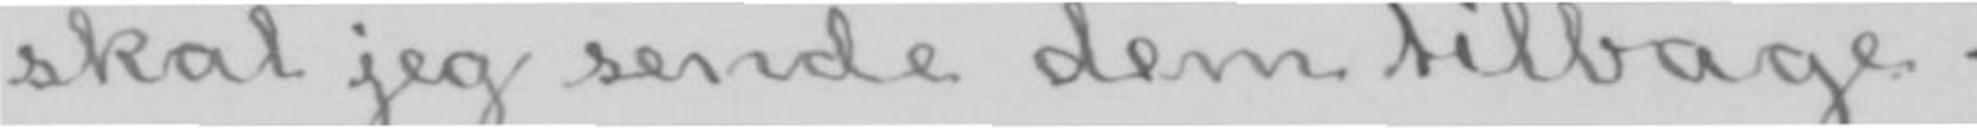skal jeg sende dem tilbage.
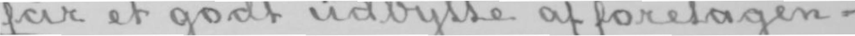får et godt udbytte af foretagen-
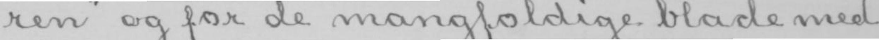ren» og for de mangfoldige blade med
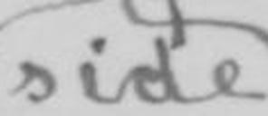side
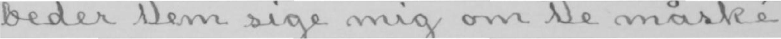beder Dem sige mig om De måské
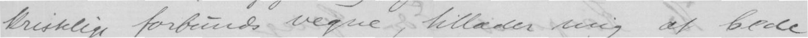dristelige forbunds vegne, tillader mig at bede
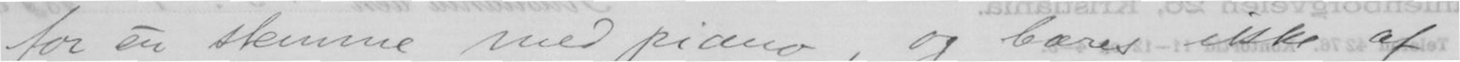for en stemme med piano, og bæres ikke af
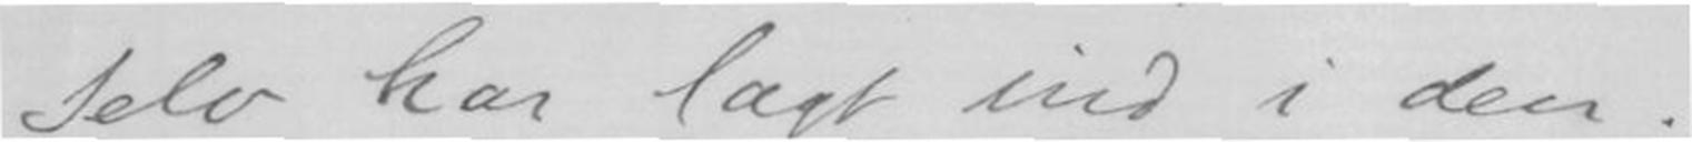selv har lagt ind i den.
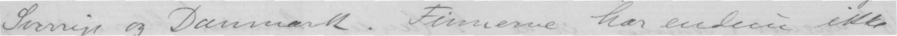Sverige og Danmark. Finnerne har endnu ikke
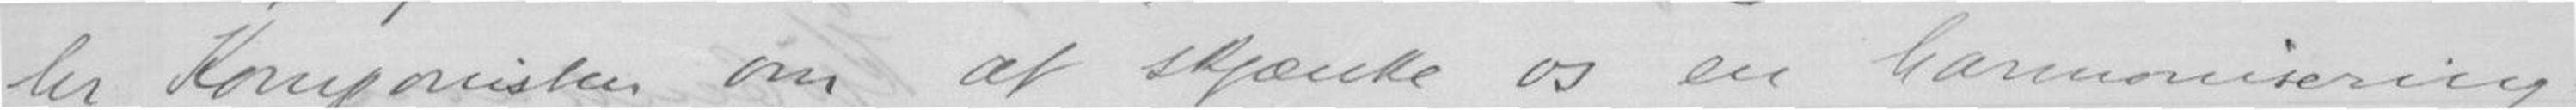hr Komponisten om at skjænke os en harmonisering
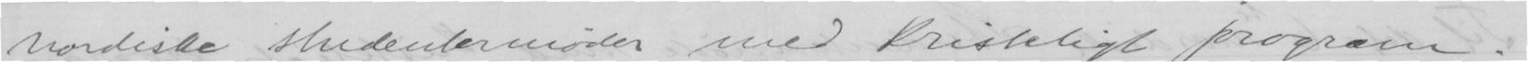nordiske studentermøder med Kristeligt program.
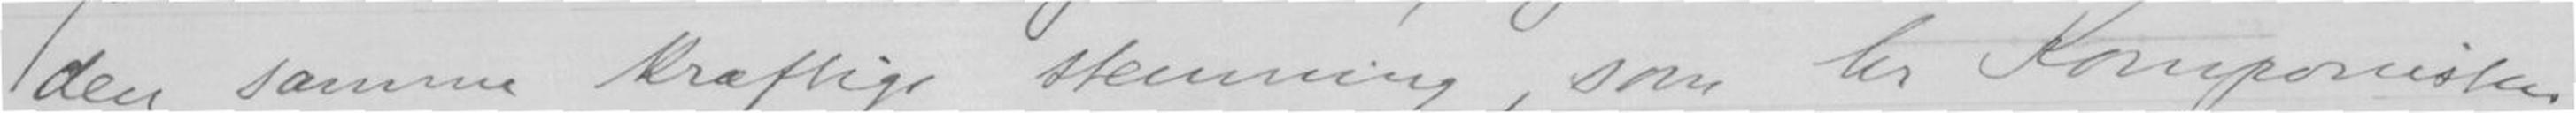den samme kraftlige stemming, som hr Komponisten
